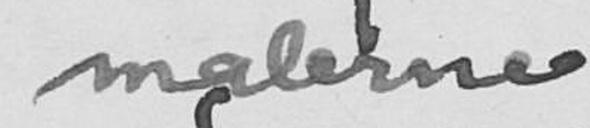malerne
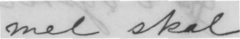mel skal
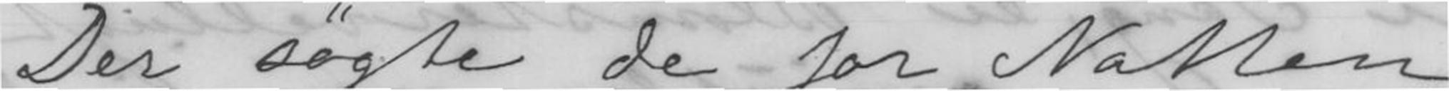Der søgte de for Natten
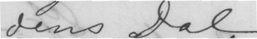dens Dal,
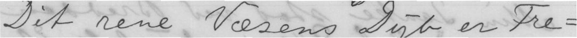Dit rene Væsens Dyb er Fre-
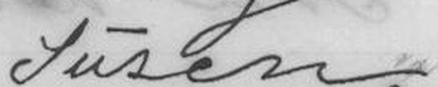Susen.
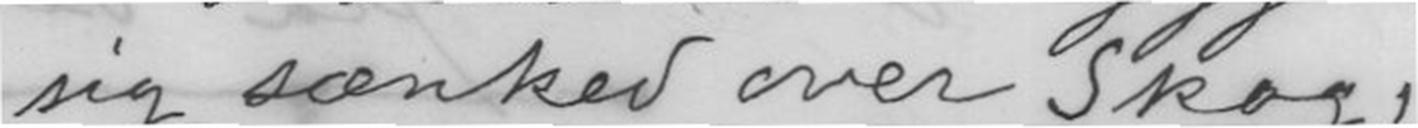sig sænked over Skog,
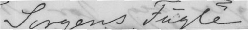Sorgens Fugle
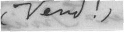(Vend!)
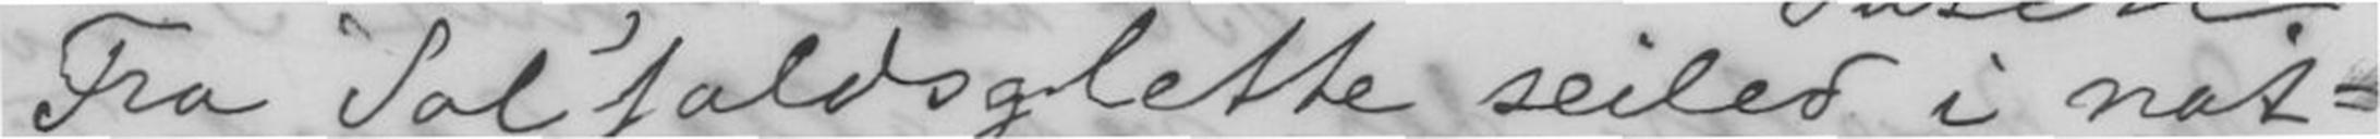Fra Sol'faldsglette seiled i nat-
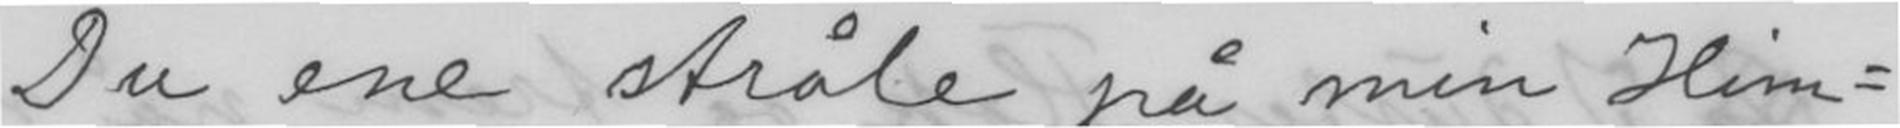- Du ene stråle på min Him-
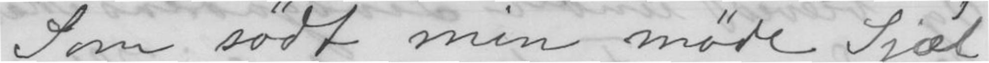Som sødt min møde Sjæl
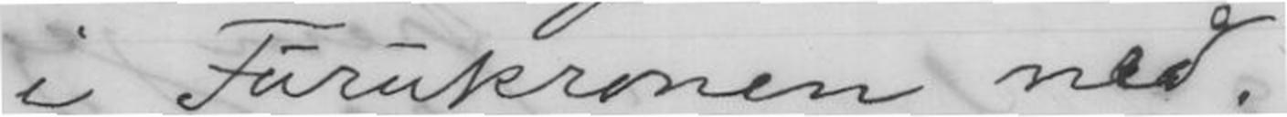i Furukronen ned.
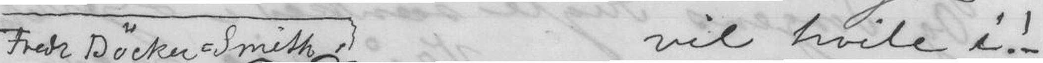Fredr Döcker=Smith vil hvile i!
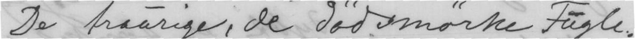De traurige, de dødsmørke Fugle.
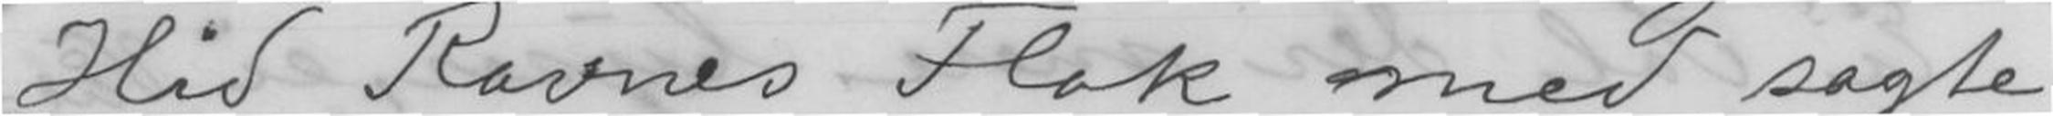Hid Ravnes Flok med sagte
